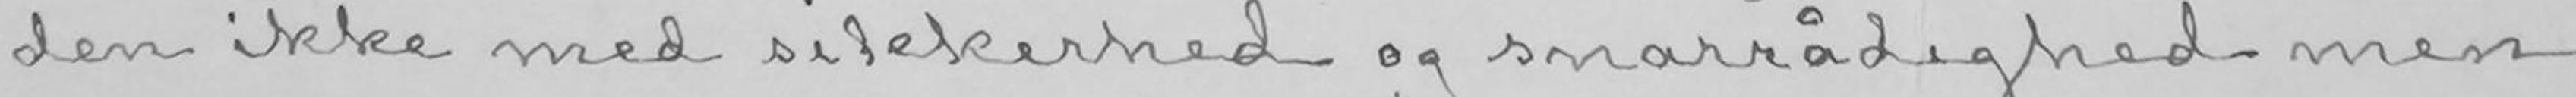den ikke med sikkerhed og snarrådighed men
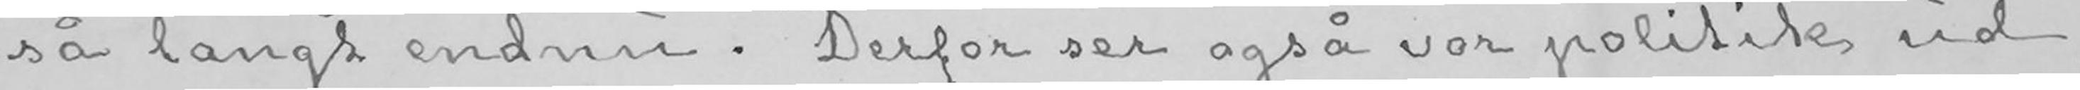så langt endnu. Derfor ser også vor politik ud,
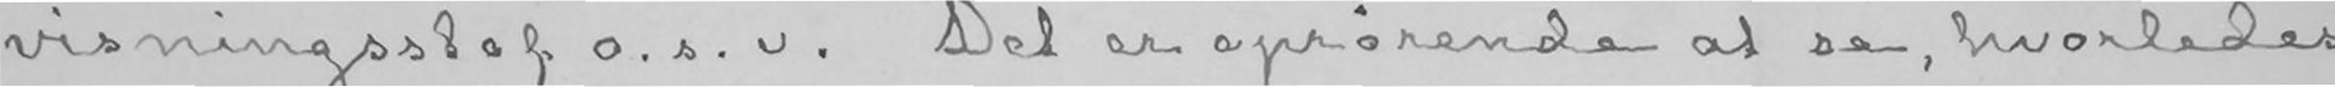visningsstof o. s. v. Det er oprørende at se, hvorledes
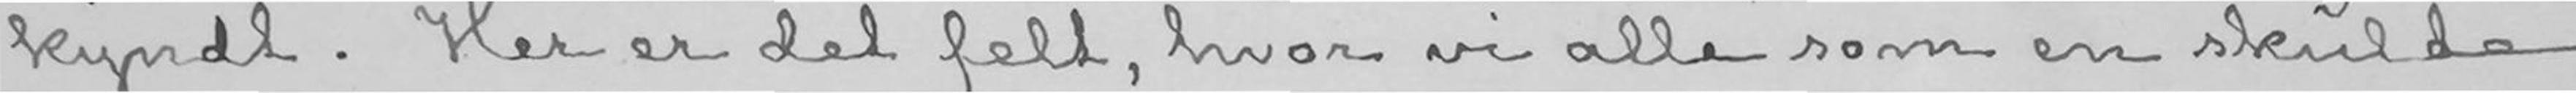kyndt. Her er det felt, hvor vi alle som en skulde
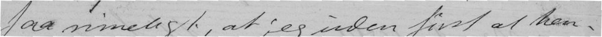saa rimeligt, at jeg uden først at hen-
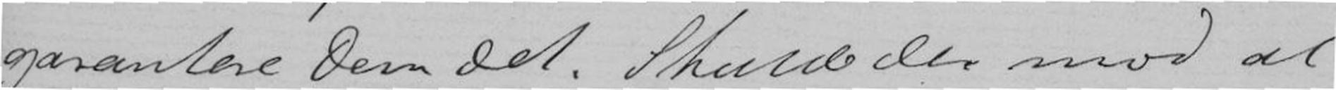garantere Dem det. Skulde der mod al
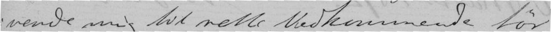vende mig til rette Vedkommende tør
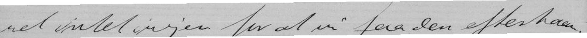vel intet ivejen for at vi saa den efterhaan-
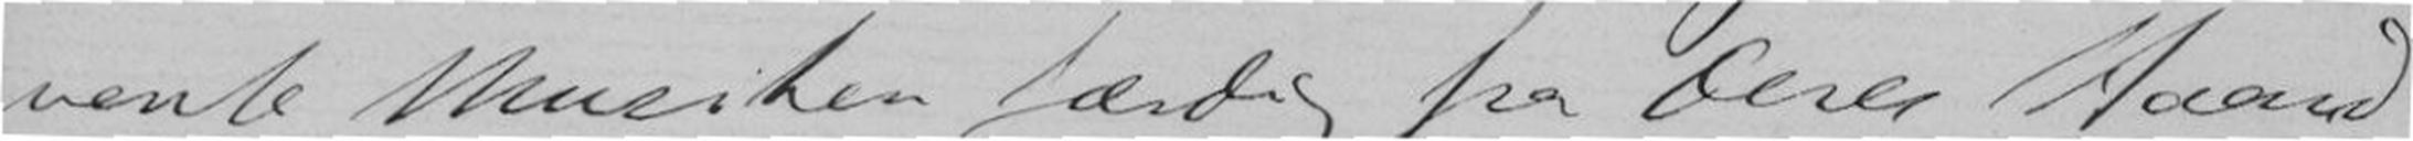vente Musiken færdig fra Deres Haand
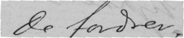De fordrer,
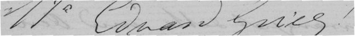Til Edvard Grieg!
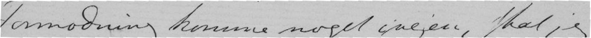Formodning komme noget ivejen, skal jeg
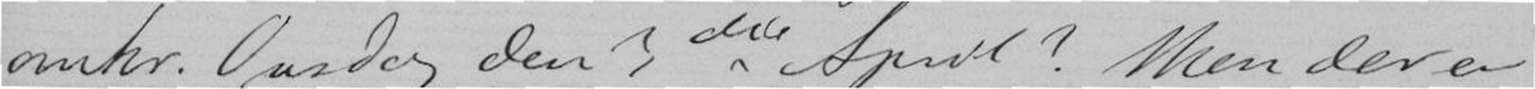omkr. Onsdag den 3 die April ? Men der er
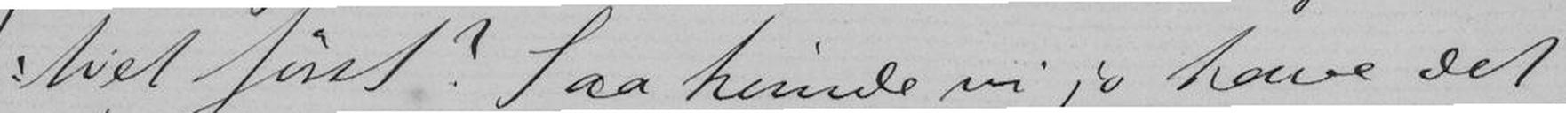tiet først? Saa kunde vi jo have det
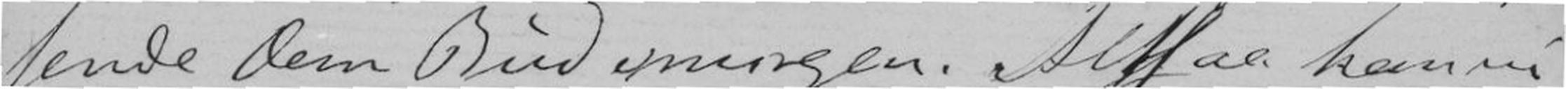sende Dem Bud imorgen. Altsaa kan vi
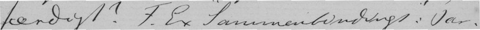færdigt ? F. Ex. "Sammenbindings": Par-
